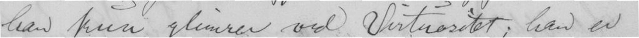han kun glimrer ved Virtuositet; han er
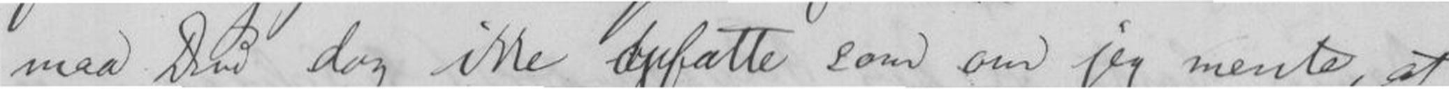maa Du dog ikke opfatte som om jeg mente, at
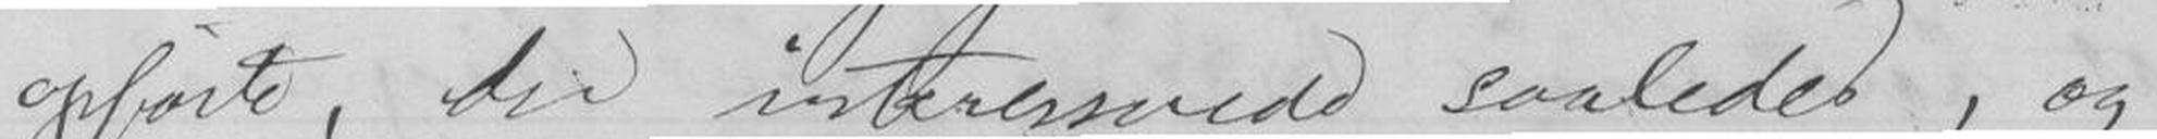opførte, der interesserede saaledes, og
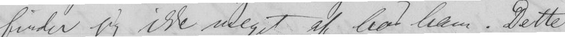finder jeg ikke meget af hos ham. Dette
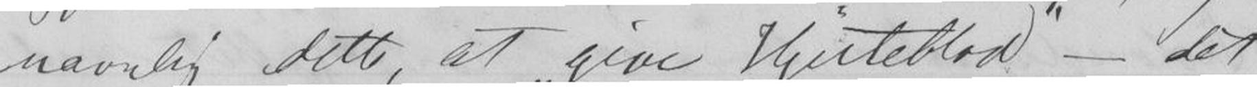navnlig dette, at give Hjerteblod - det
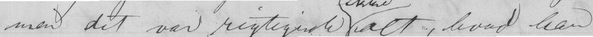men det var rigtignok alt, hvad han
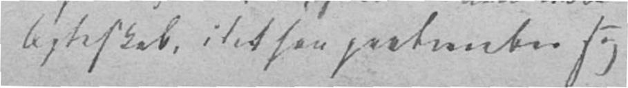Ægteskab, idet han paaberaaber sig
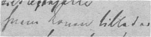hvem Loven tillader
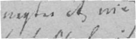nægter at vie
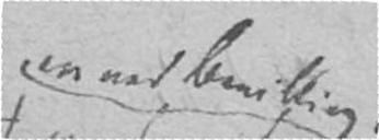en ved Bevilling
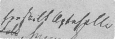fraskilt Ægtefælle
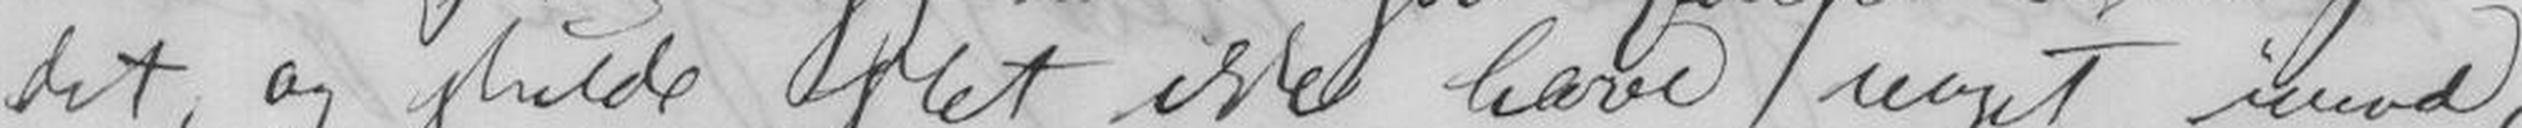det, og skulde slet ikke have noget imod,
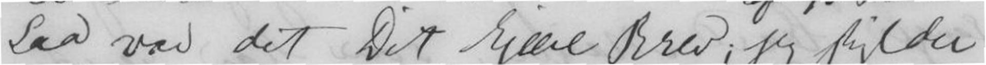Saa var det Dit kjære Brev; jeg skylder
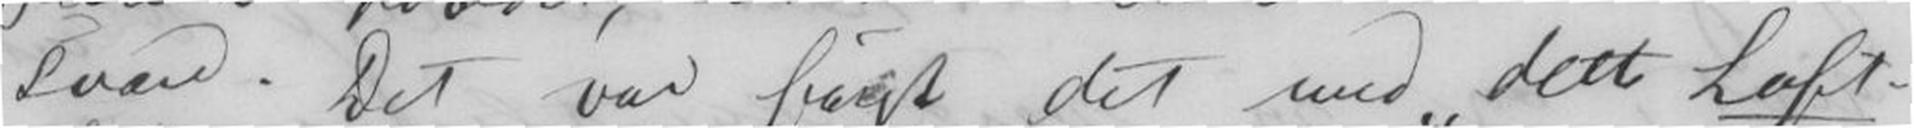Svare. Det var først det med dette Loft-
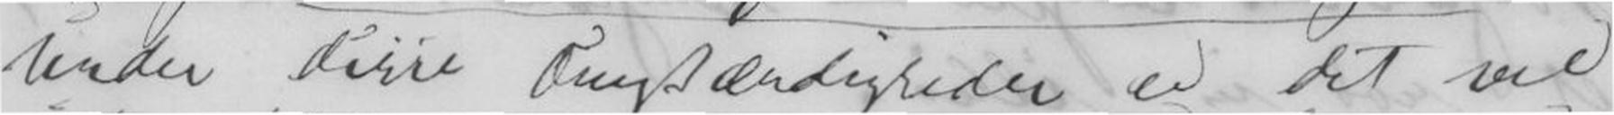Under disse Omstændigheder er det vel
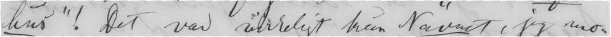hus! Det var virkeligt kun Navnet, jeg mo-
